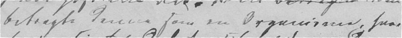betragte denne som en Organisme, hvor
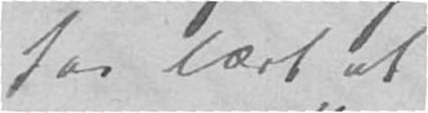har lært at
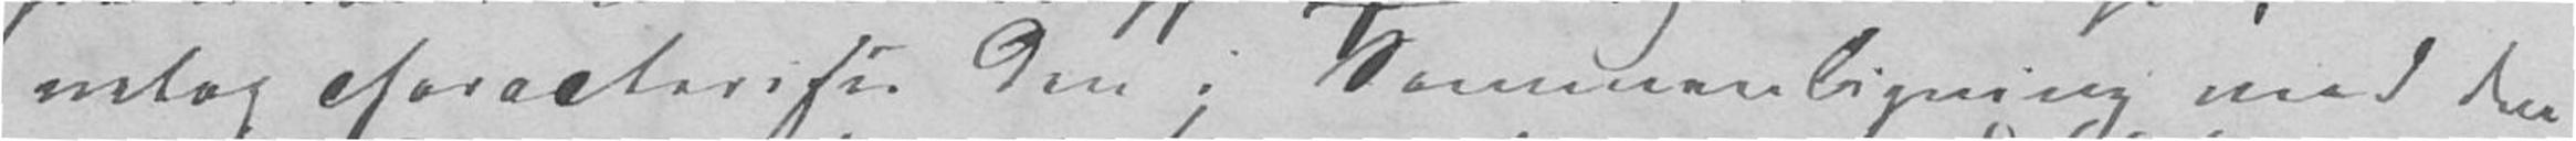netop characteriser den i Sammenligning med den
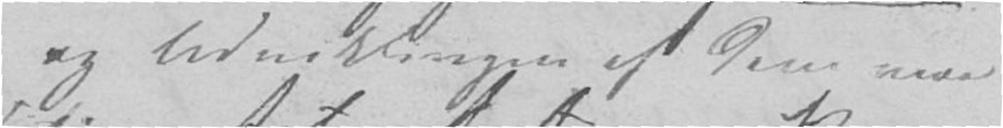og Udviklingen af dem maa
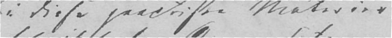i disse practiske Materier
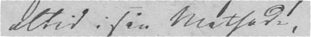altid i sin Methode,
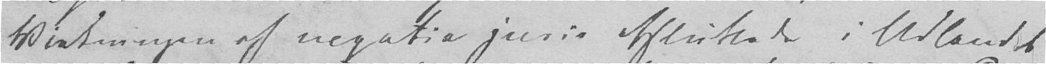Virkningen af negatio juris afsluttede i Udlandet
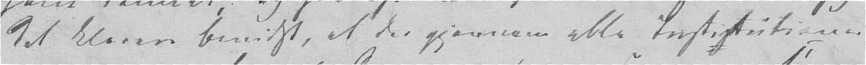det klarere bevidst, at der gjennem alle Institutioner
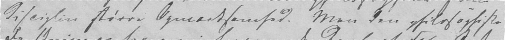Disciplin større Opmærksomhed. Men den philosophiske
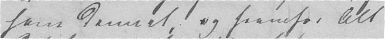have dannet, og fremfor Alt
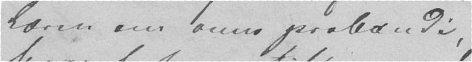Læren om onus probandi,
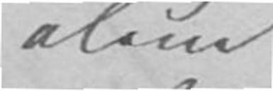alene
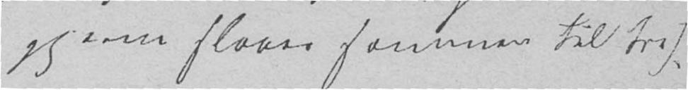gjerne slaaes sammen til tre).
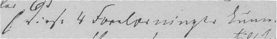( Disse 4 Forelæsninger kunne
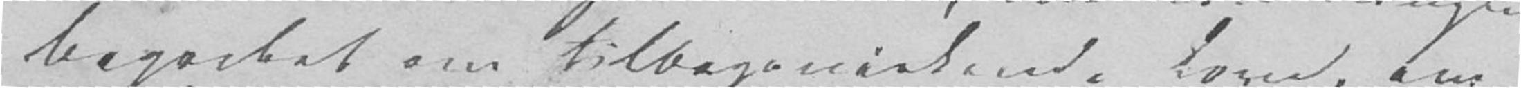Begrebet om tilbagevirkende Love, om
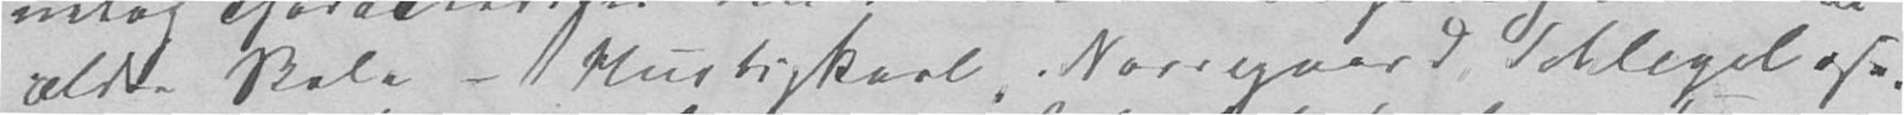ældre Skole - Hurtigkarl, Nørregaard, Schlegel osv.
